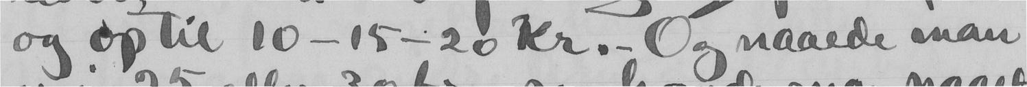og optil 10 - 15 - 20 Kr. - Og naaede man
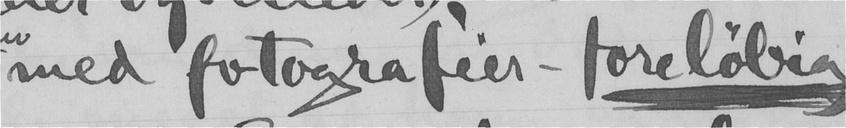med fotografier - foreløbig -;
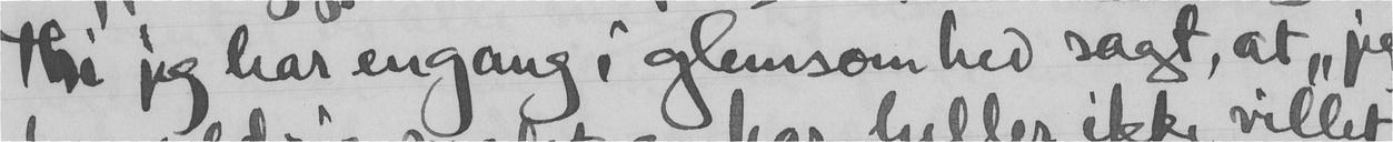thi jeg har engang i glemsomhed sagt, at "jeg
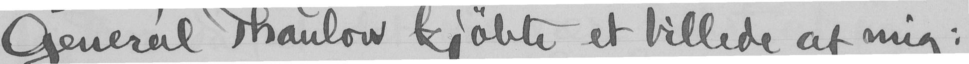General Thaulow kjøbte et billede af mig:
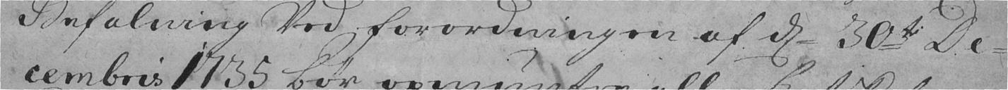Befalning Ved forordningen af d 30te De-
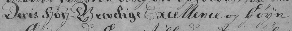Deris Høy-Grevelige Excellence og Høye
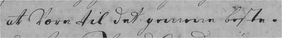at være til det gemene beste.
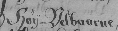Høy-Velbaarne
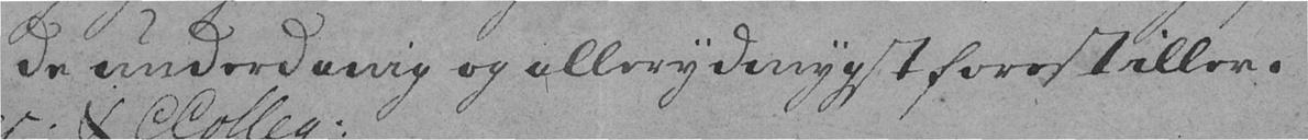de underdanig og allerydmygst forestiller.
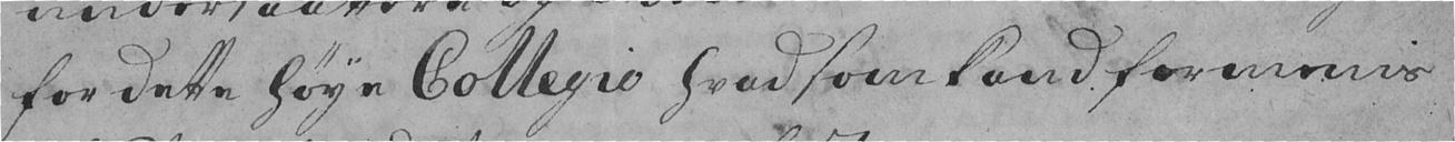for dette høye Collegio hvad som kand formenis
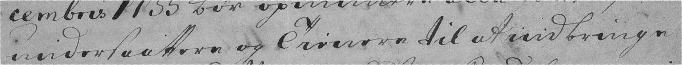undersaattere og Tienere til at indbringe
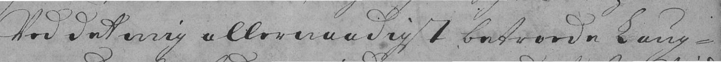Ved det mig allernaadigst betroede Laug-
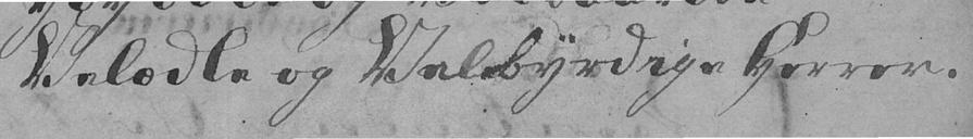Velædle og Velbyrdige Herrer
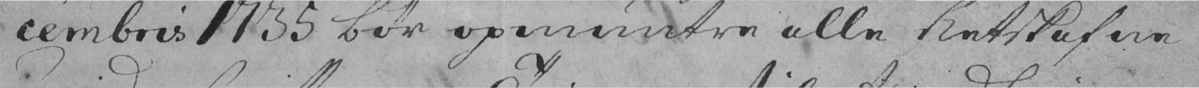cembris 1735 bør opmuntre alle Retskafne
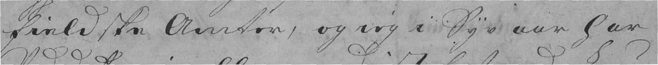fieldske Amter, og ieg i Syv aar har
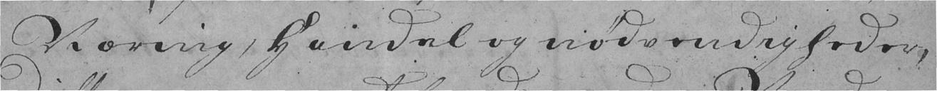Næring, handel og nødvendigheder,
